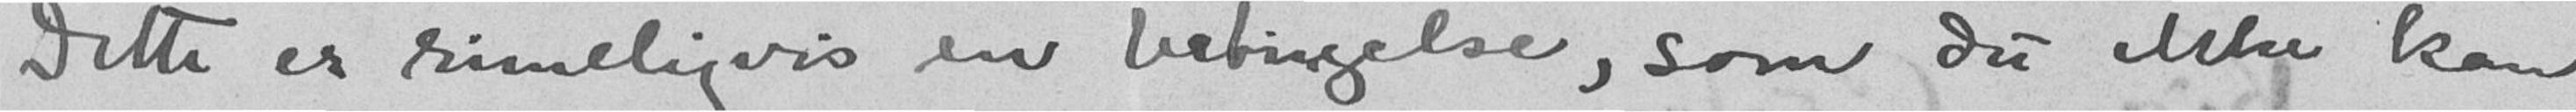Dette er rimeligvis en betingelse, som du ikke kan
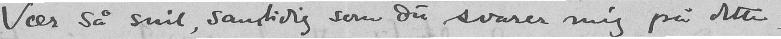Ver så snil, samtidig som du svarer mig på dette,
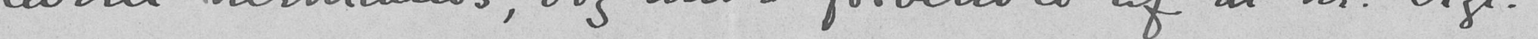leveret hertillands, dog under forbehold af at hr. Vige-
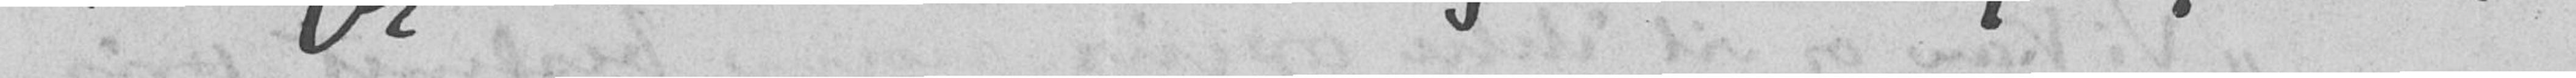- Jeg har der i de sidste uger været optaget med
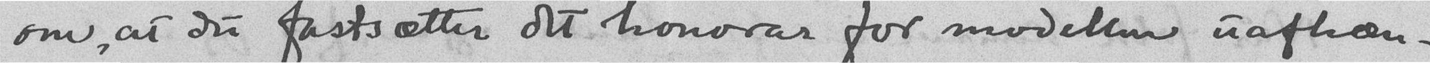om, at du fastsætter det honorar for modellen uafhæn-
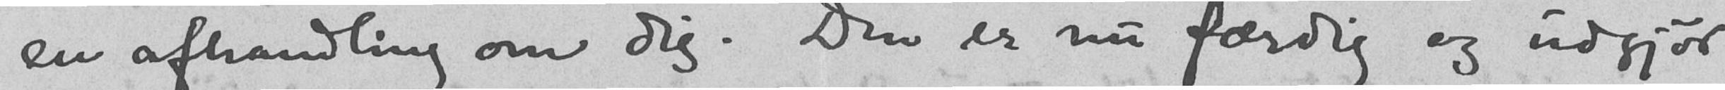en afhandling om dig. Den er nu færdig og udgjør
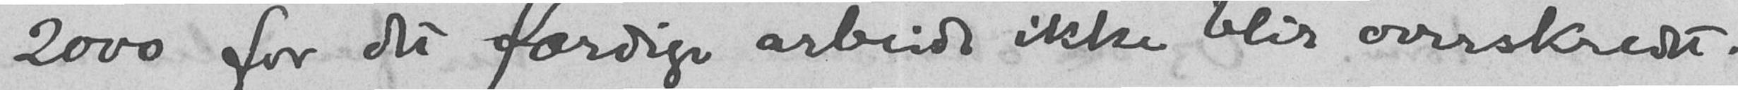2000 for det færdige arbeide ikke blir overskredet.
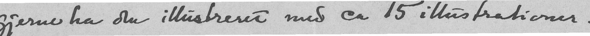gjerne ha den illustreret med ca. 15 illustrationer.
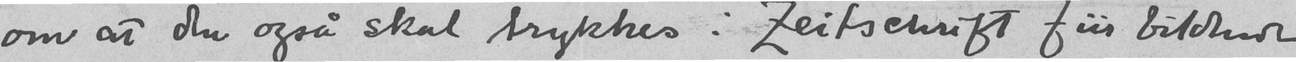om at den også skal trykkes: "Zeitschrift für bildende
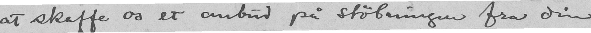at skaffe os et anbud på støbningen fra din
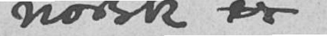norsk
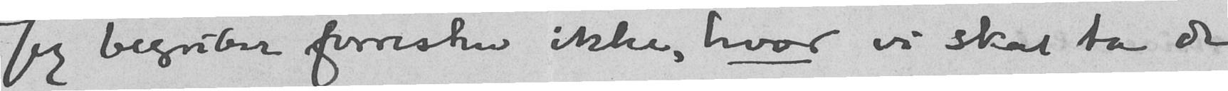Jeg begriper forresten ikke, hvor vi skal ta de
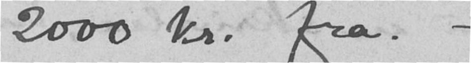2000 kr. fra. -
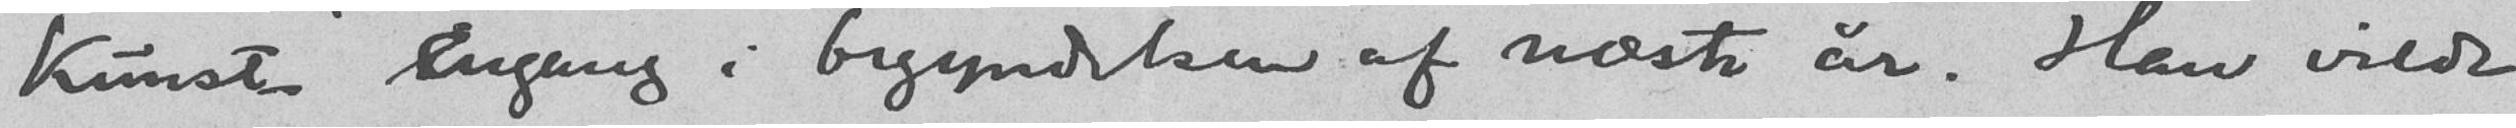Kunst" engang i begyndelsen af næste år. Han vilde
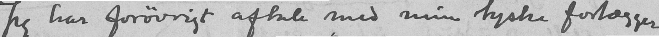Jeg har forøvrigt aftale med min tyske forlægger
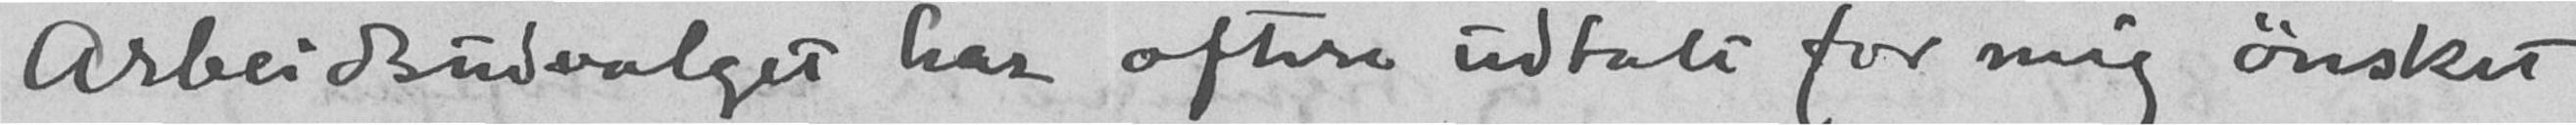Arbeidsudvalget har oftere udtalt for mig ønsket
